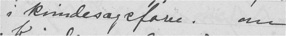i kvindesagsforn. om
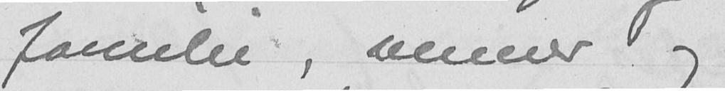familie, venner og
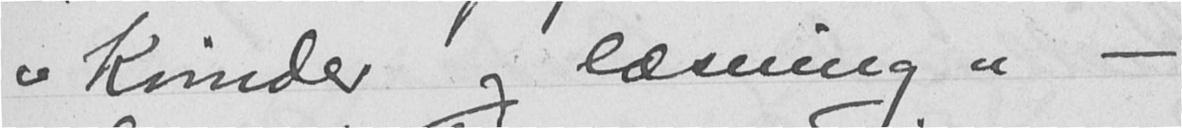"Kvinder og læsning" -
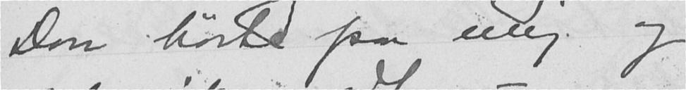Don hørte paa mig og
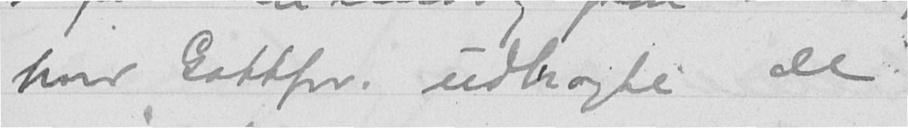hvor Gottfr. udbragte de
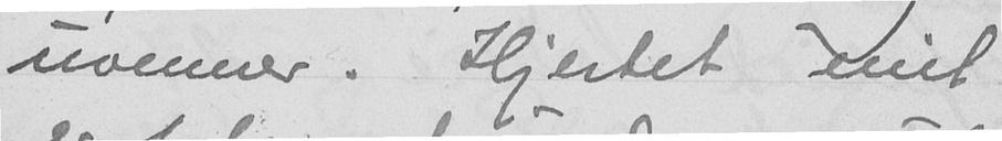uvenner. Hjertet mit
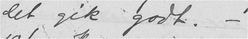det gik godt. -
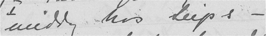middag hos Seips -
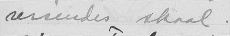reisendes skaal.
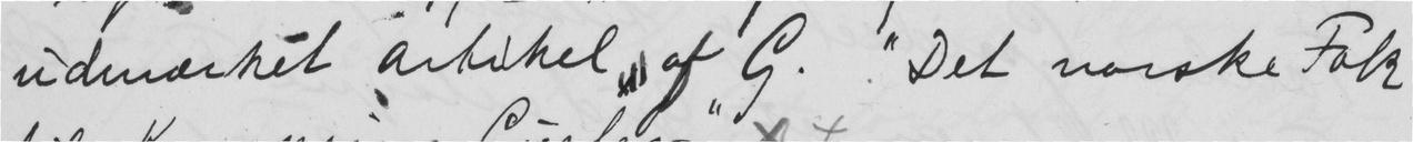udmærket artikel af G. "Det norske Folk
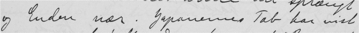og Enden nær. Japanernes Tab har vist
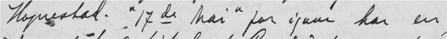Hognestad. "17de Mai" for igaar har en
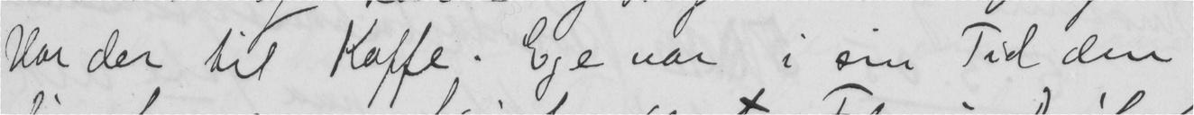Var der til Kaffe. Ege var i sin Tid den
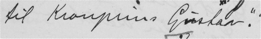til Kronprins Gustav."
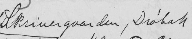Skrivergaarden, Drøbak
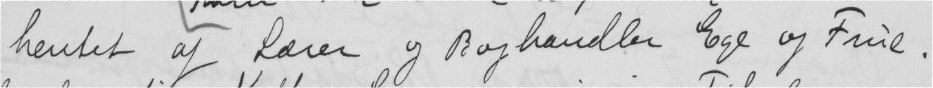hentet af Lærer og Boghandler Ege og Frue.
